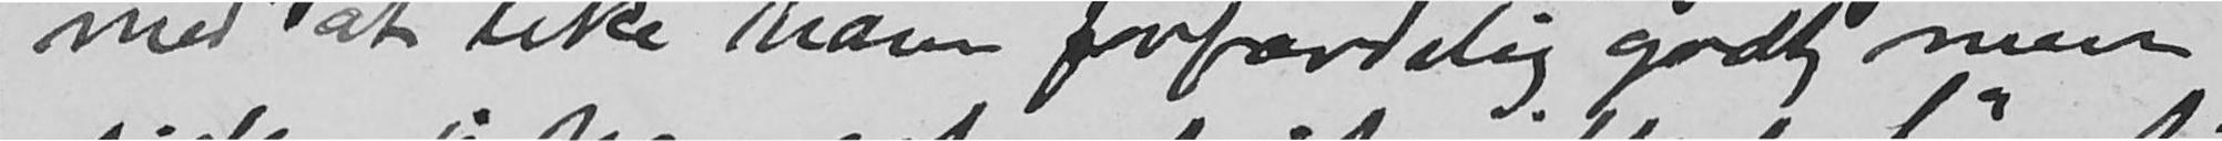med at like ham forfærdelig godt, men
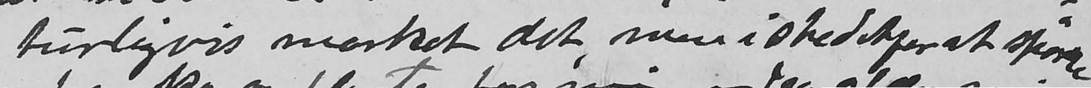turligvis merket det, men i stedetfor at spørre
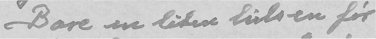Bare en liten hilsen før
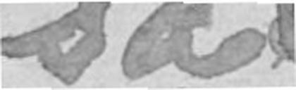så
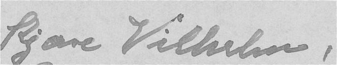Kjære Vilhelm,
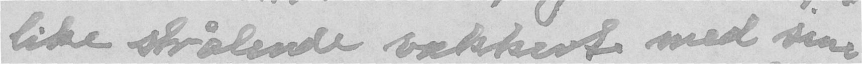like strålende vakkert med sine
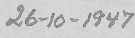26-10-1947
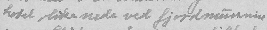hotel, like nede ved fjordmunnin-
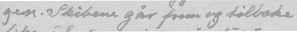gen. Skibene går frem og tilbake
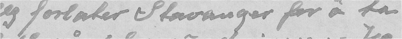jeg forlater Stavanger for å ta
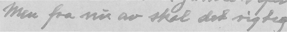Men fra nu av skal det rigtig
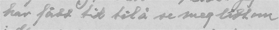har fått tid til å se meg litt om
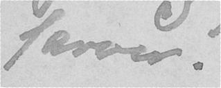farver.
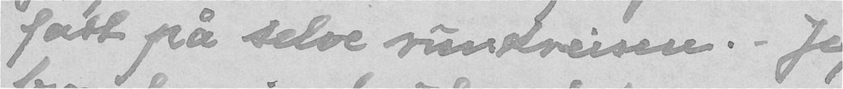fatt på selve rundreisen. - Jeg
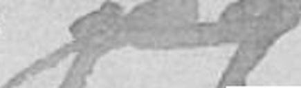jeg
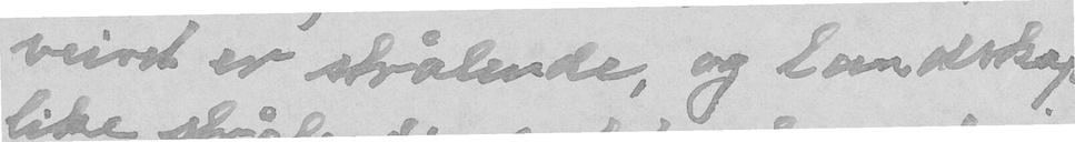veiret er strålende, og landskapet
